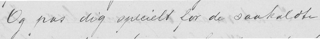Og pas dig specielt for de saakaldte
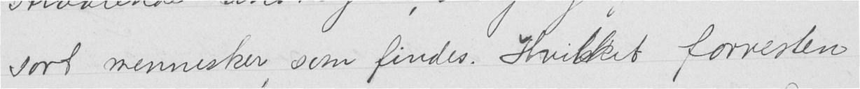sort mennesker, som findes. Hvilket forresten
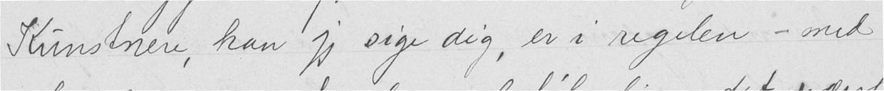Kunstnere, kan jeg sige dig, er i regelen - med
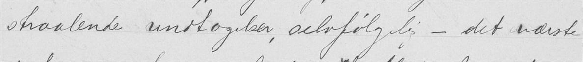straalende undtagelser, selvfølgelig - det værste
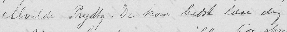Alvilde Prydtz. De kan bedst lære dig
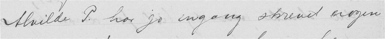Alvilde P. har jo engang skrevet nogen
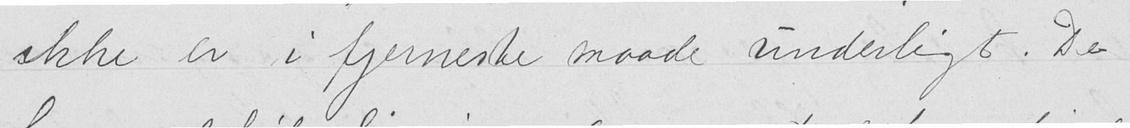ikke er i fjerneste maade underligt. De
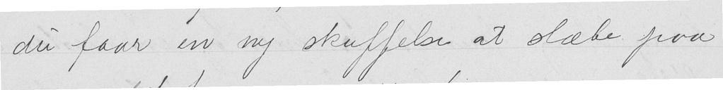du faar en ny skuffelse at slæbe paa
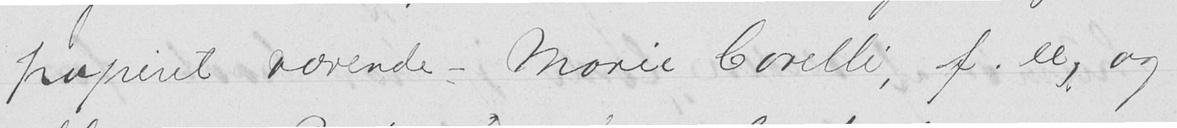papiret værende - Marie Corelli, f.ex., og
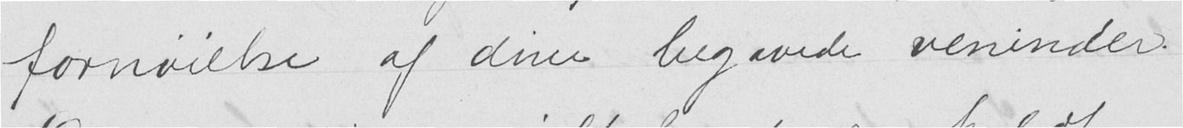fornøielse af dine begavede veninder.
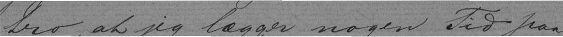tro, at jeg lægger nogen Tid paa
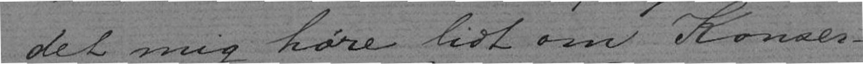det mig høre lidt om Konser-
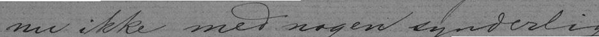nu ikke med nogen synderlig
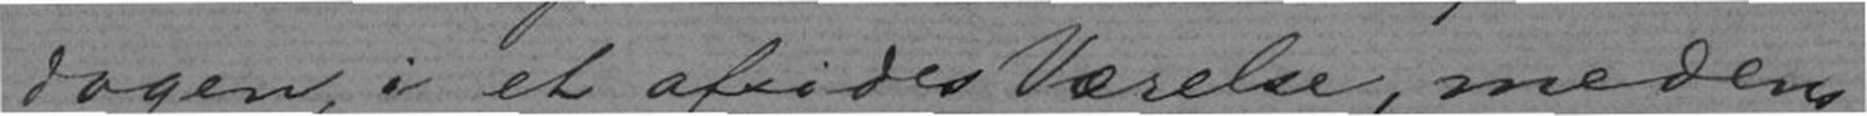dagen, i et afsides Værelse, medens
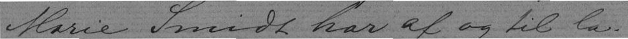Marie Smidt har af og til la-
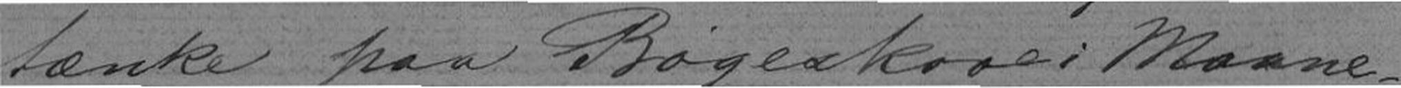tænke paa Bøgeskove; Maane-
